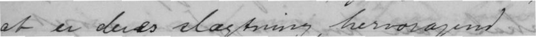at er deres slægtning "hervoragend
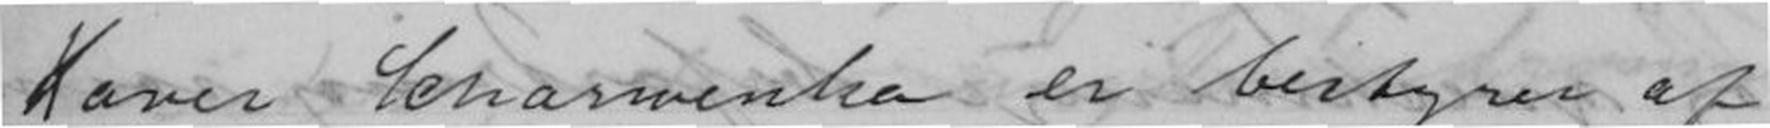Xaver Scharwenka er bestyrer af
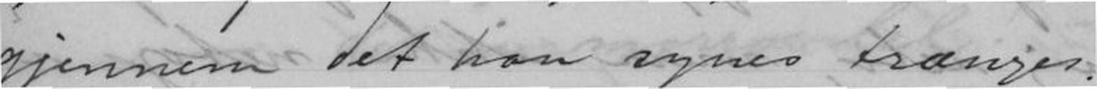gjennem det han synes trænges.
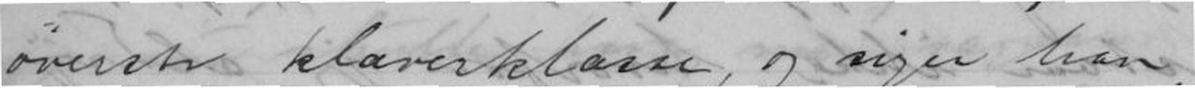øverste klaverklasse, og siger han,
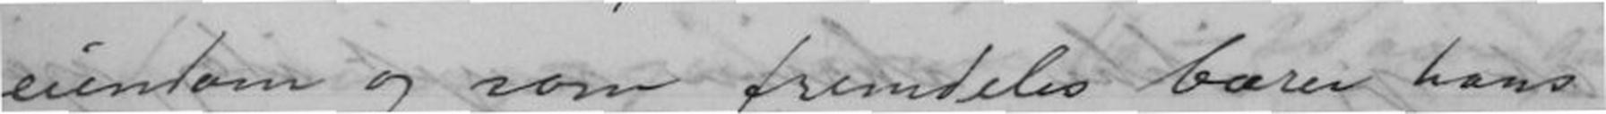eiendom og som fremdeles bærer hans
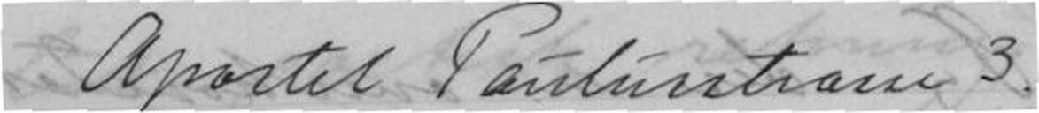Apostel Paulusstrasse 3.
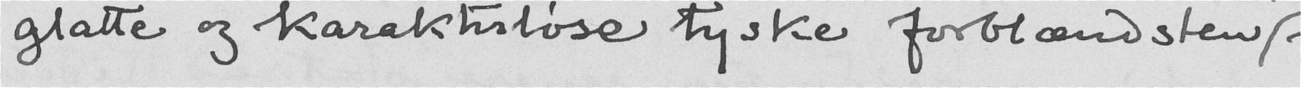glatte og karakterløse tyske "forblændsten"
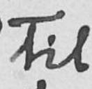til
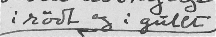i rødt og i gullt
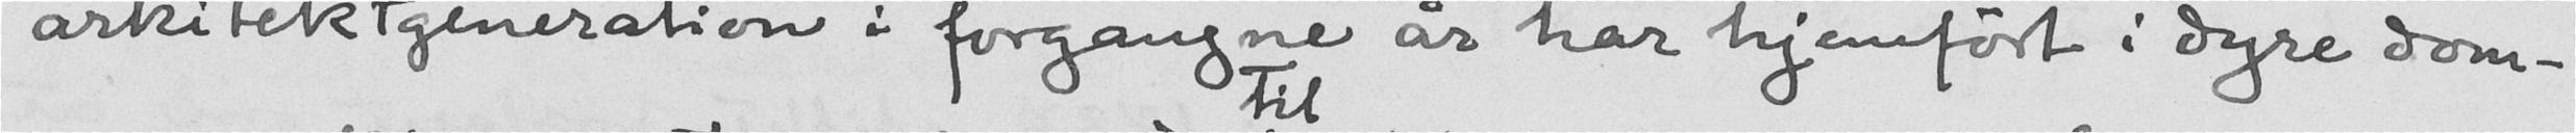arkitektgeneration i forgangne år har hjemført i dyre dom-
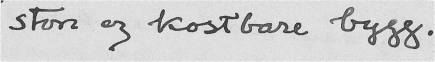store og kostbare bygg.
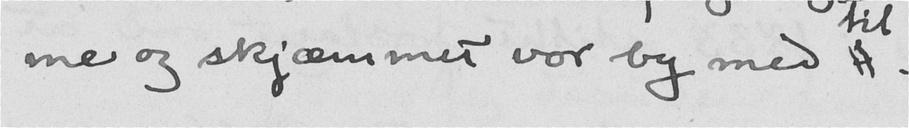me og skjæmmet vor by med
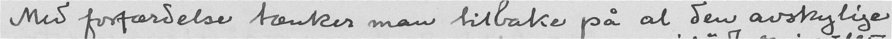Med forfærdelse tænker man tilbake på al den avskylige
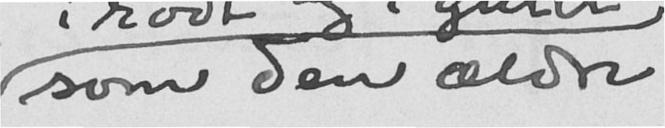som den ældre
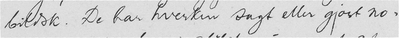bildsk. De har hverken sagt eller gjort no-
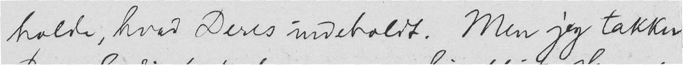holde, hvad Deres indeholdt. Men jeg takker
